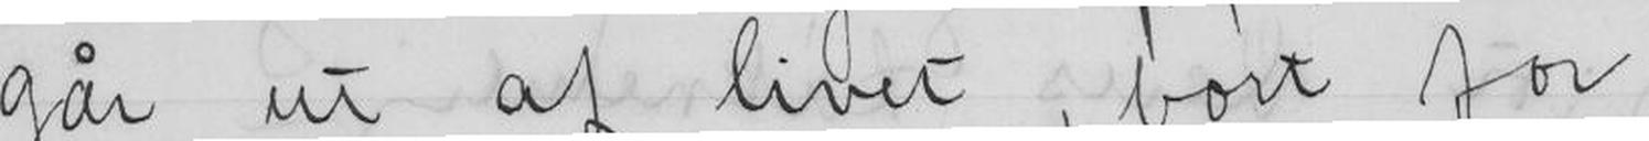går ut af livet, bort for
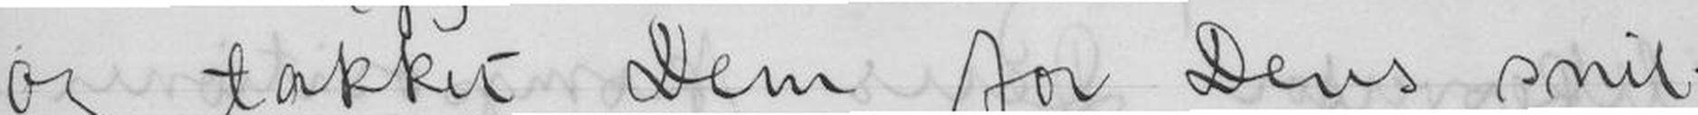og takket Dem for Deres snil-
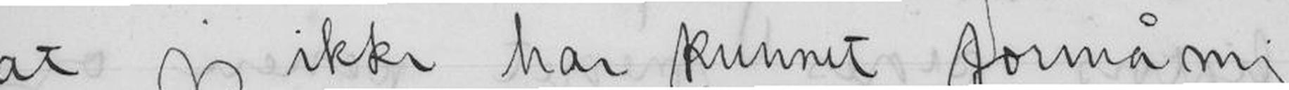at jeg ikke har kunnet formå mig
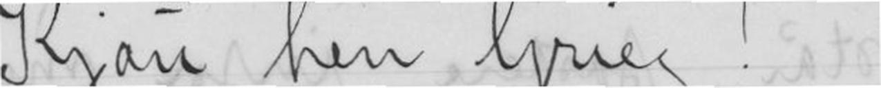Kjære herr Grieg!
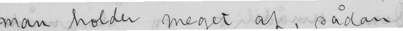man holder meget af, sådan
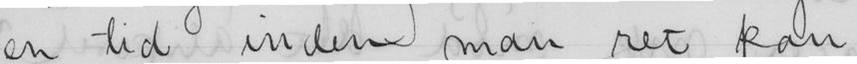en tid inden man ret kan
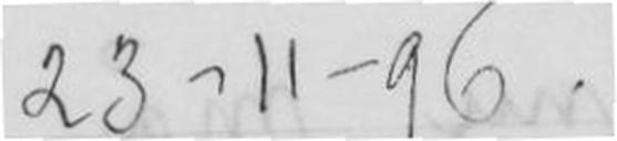23 -11- 96.
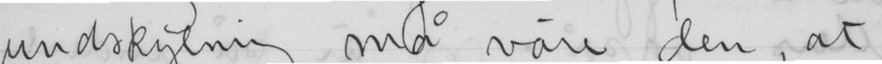undskyldning må väre den, at
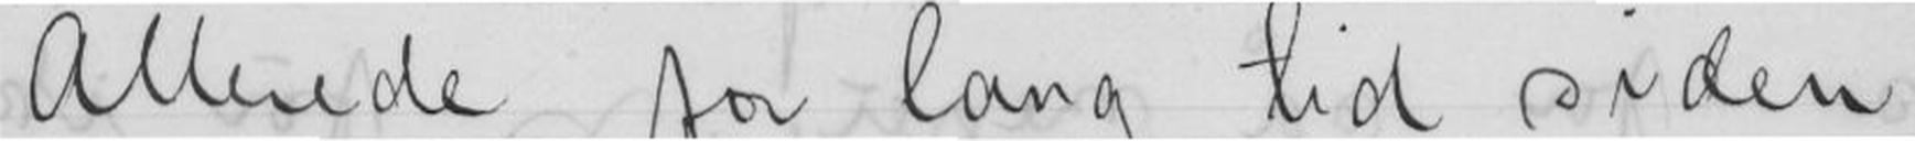Allerede for lang tid siden
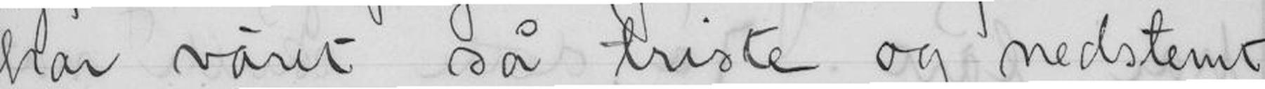har väret så triste og nedstemt
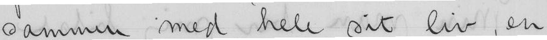sammen med hele sit liv, en,
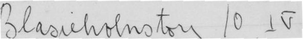Blasieholnstorg 10, IV
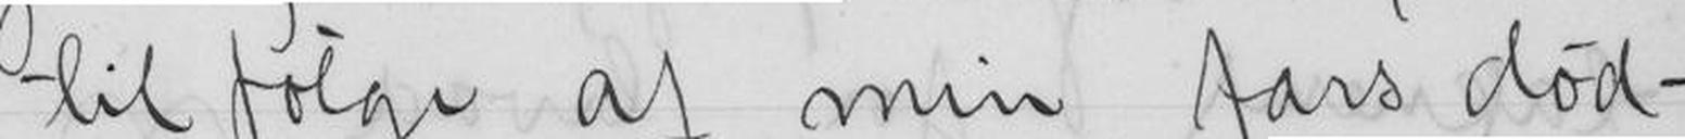til følge af min fars død -
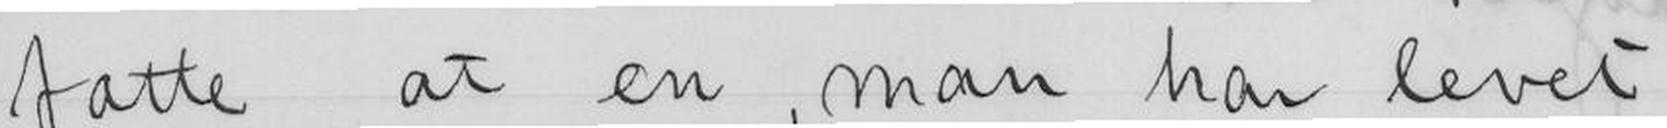fatte at en, man har levet
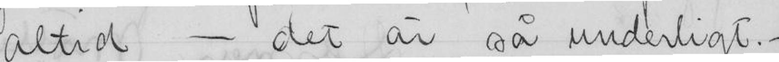altid - det är så underligt.-
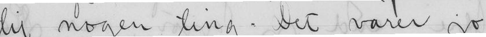til nogen ting. Det varer jo
